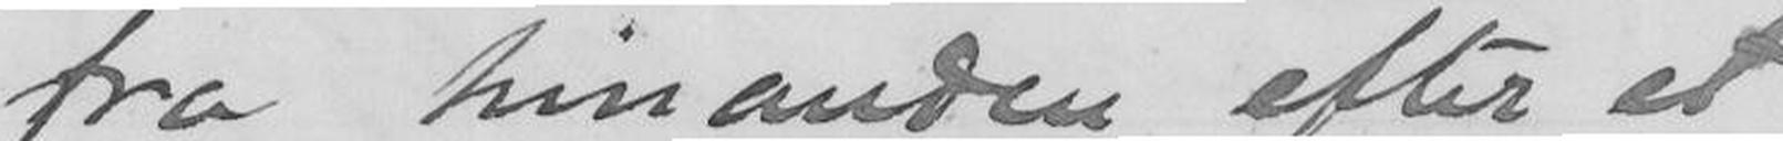fra hinanden efter et
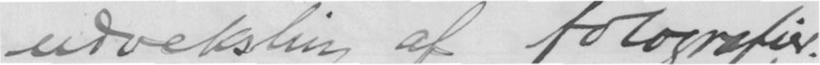udveksling af fotografier.
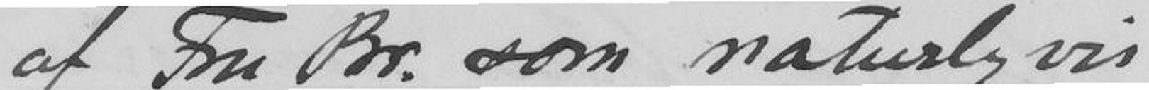af Fru Br. som naturligvis
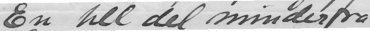En hel del minder fra
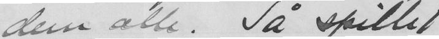dem alle. Så spillet
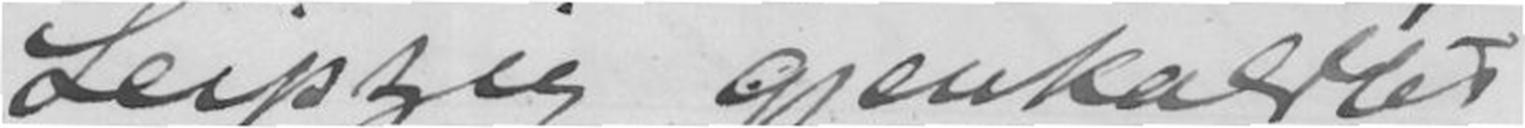Leipzig gjenkalded
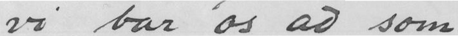vi bar os ad som
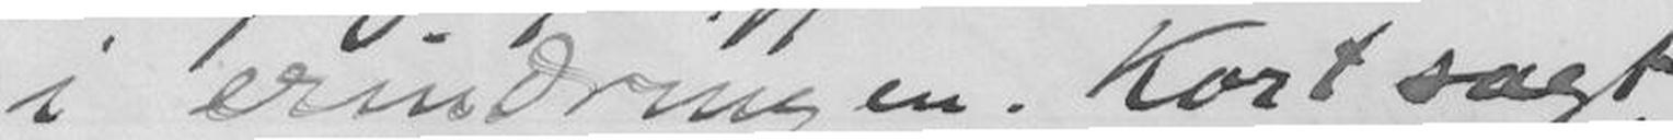i erindringen. Kort sagt,
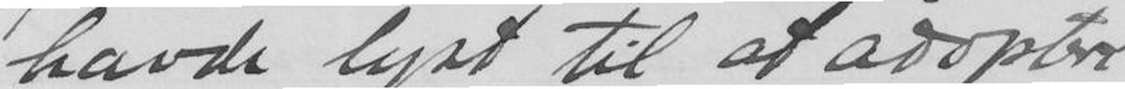havde lyst til at adoptere
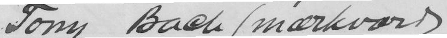Tony Bach (mærkværdig
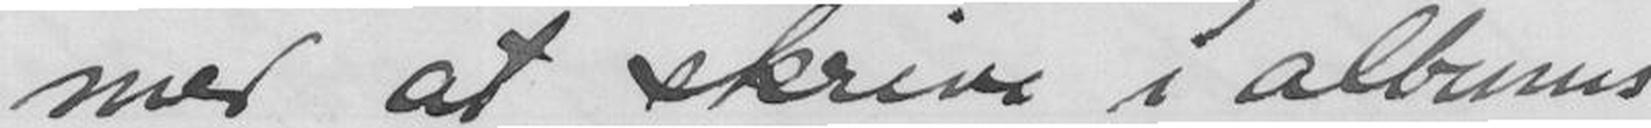med at skrive i albums
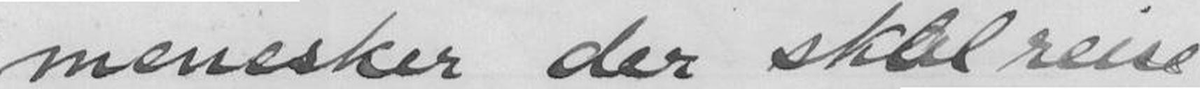menesker der skal reise
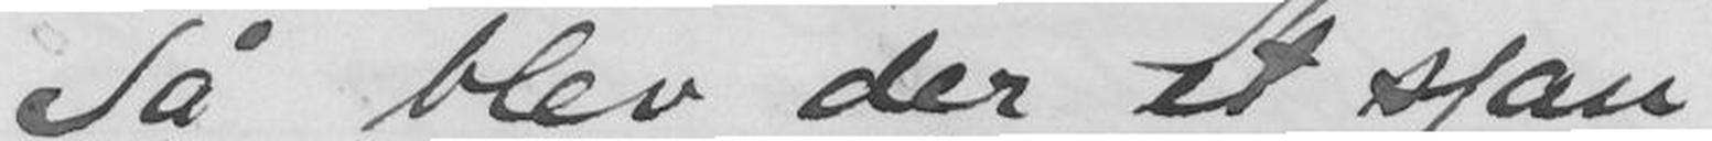Så blev der et sjau
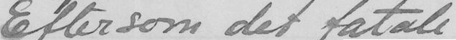Eftersom det fatale
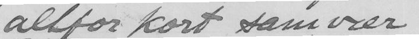altfor kort samvær.
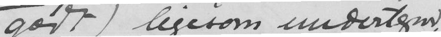godt) ligesom undertegnede
